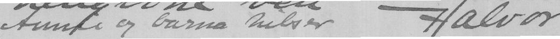Annie og barna hilser Halvor
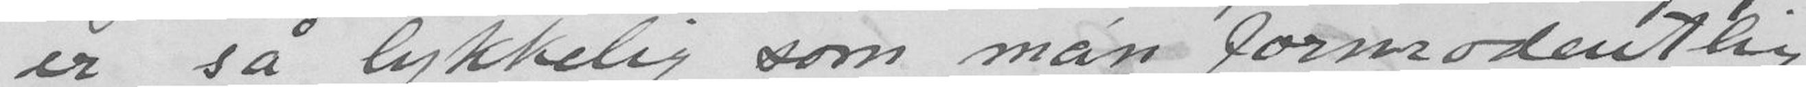er så lykkelig som man formodentlig
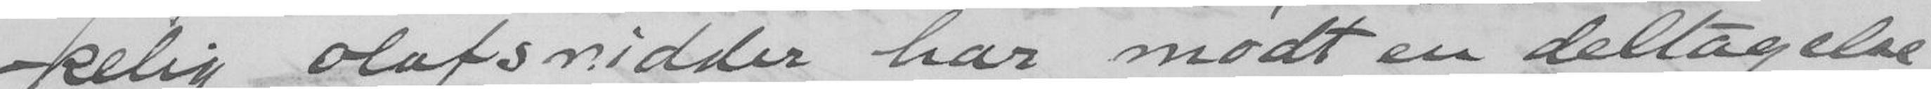elig Olafsridder har mødt en deltagelse
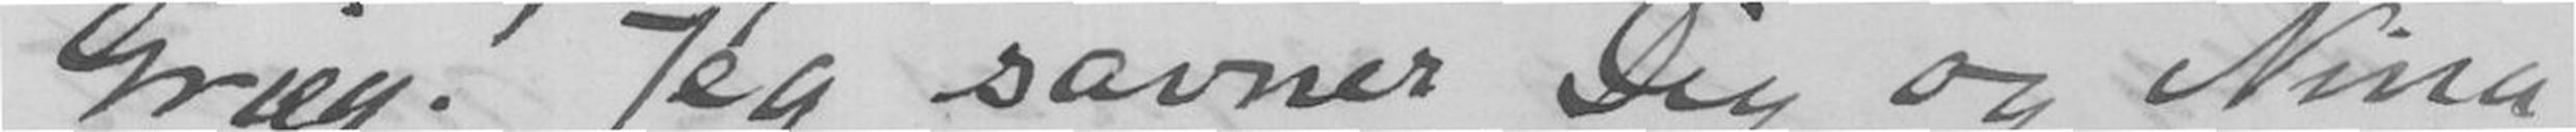Grieg ! Jeg savner Dig og Nina
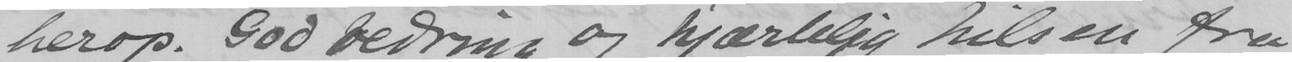herop. God bedring og hjærtelig hilsen fra
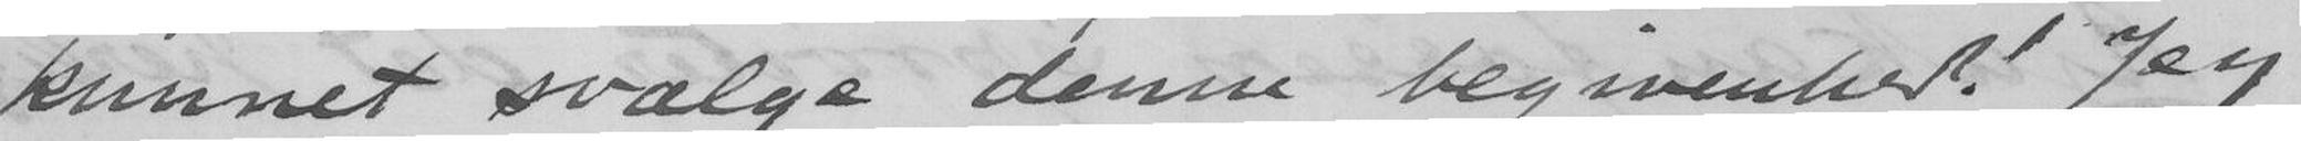kunnet svælge denne begivenhed! Jeg
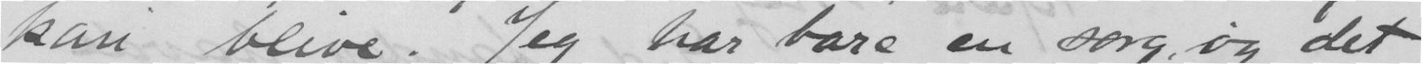kan blive. Jeg har bare en sorg, og det
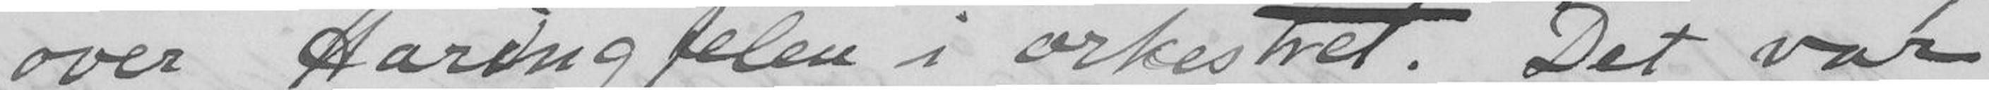over Hardingfelen i orkestret. Det var
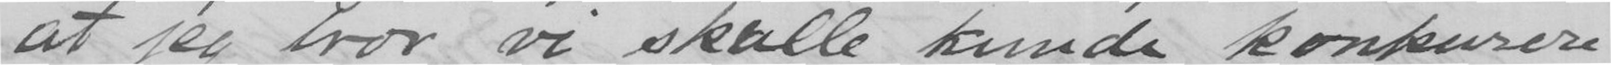at jeg tror vi skulle kunde konkurere
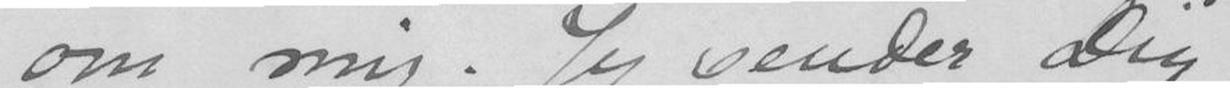om mig. Jeg sender Dig
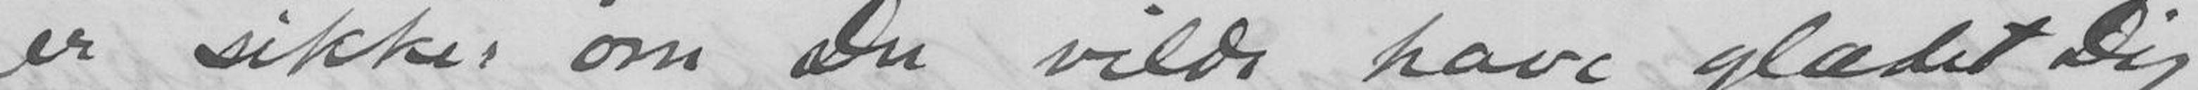er sikker om Du vilde have glædet Dig
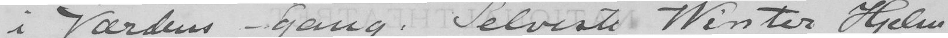i Værdens Gang. Selveste Winter Hjelm
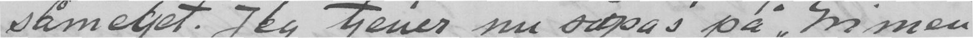såmeget. Jeg tjener nu såpass på Grimen
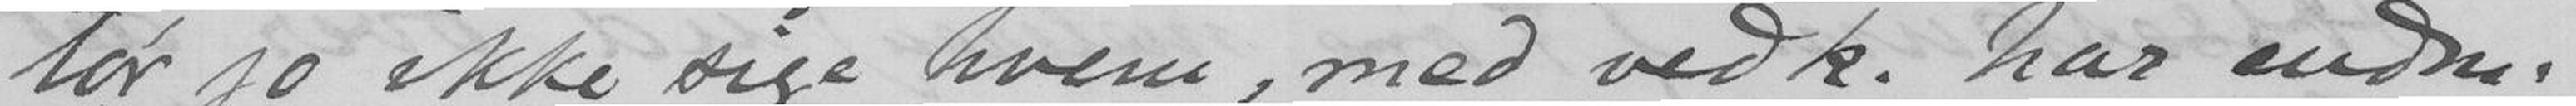tør jo ikke sige hvem, men vedk. har endnu
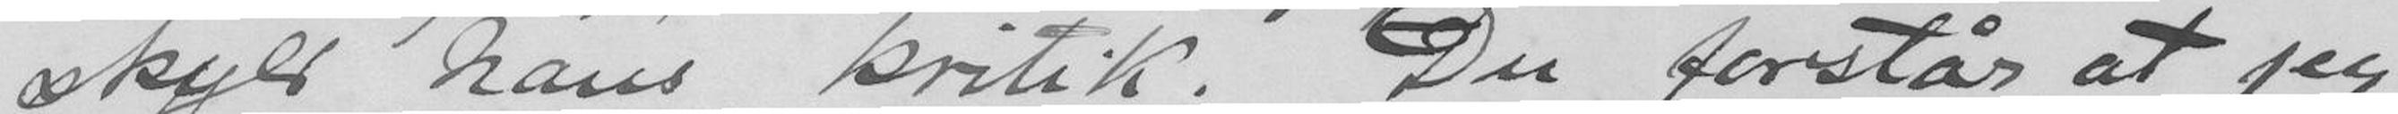skyld hans kritik. Du forstår at jeg
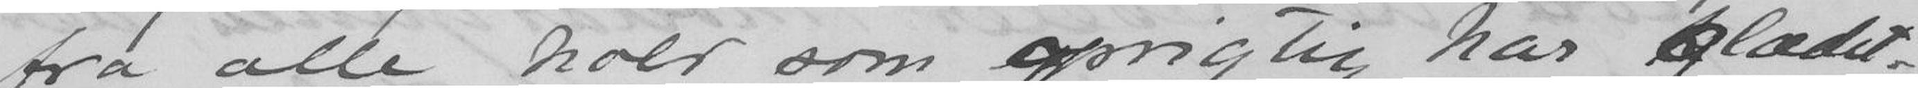fra alle hold som oprigtig har glædet
